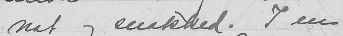nat og snakked. I en
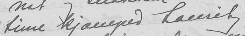time kjæmped Lauritz
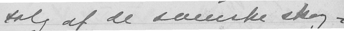salg af de svenske skog-
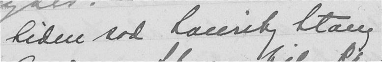Siden sad Lauritz Stang
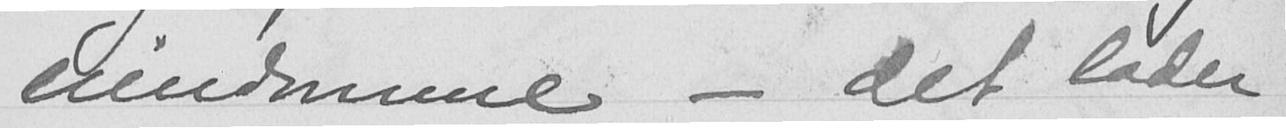eiendomme - det lader
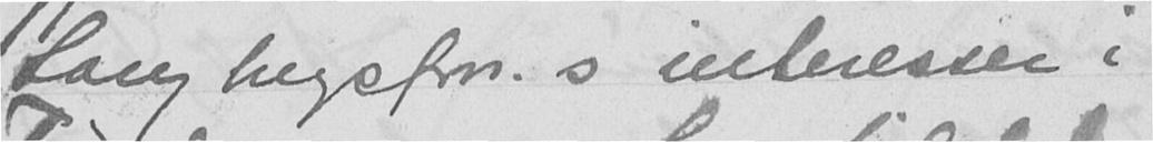Saugbrugsforn. s interesser i
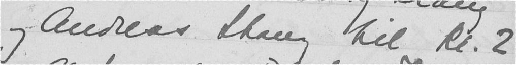og Andreas Stang til kl. 2
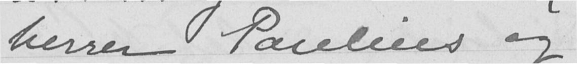herrer Parelius og
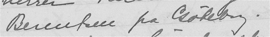Berentsen fra Gøteborg.
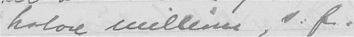halve million, s. f.
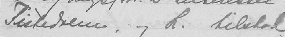Tistedalen, og L. tilstod
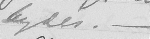byder. -
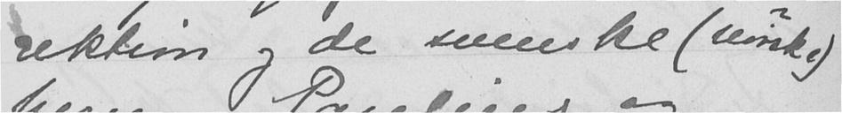rektion og de svenske (norske)
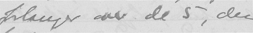forlanger over de 5, de
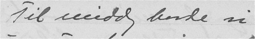Til middag havde vi
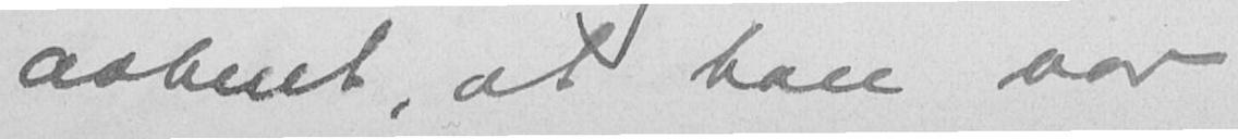aabent, at han var
